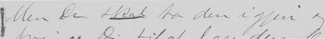Men Du skal ta den igjen og
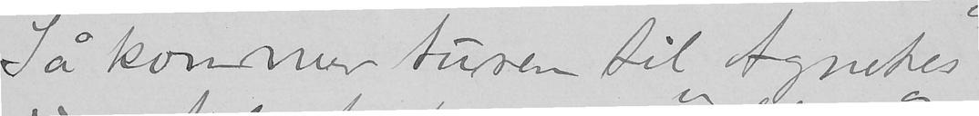Så kommer turen til "Agnetes
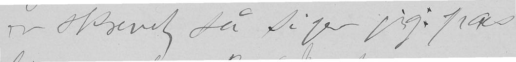er skrevet, så siger jeg: pas
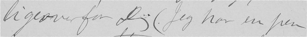ligeoverfor dig (Jeg har en pen
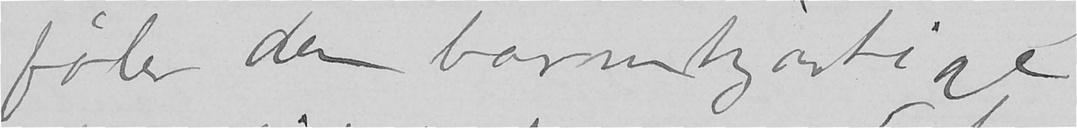føler den barmhjærtige
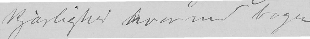kjærlighed hvormed bogen
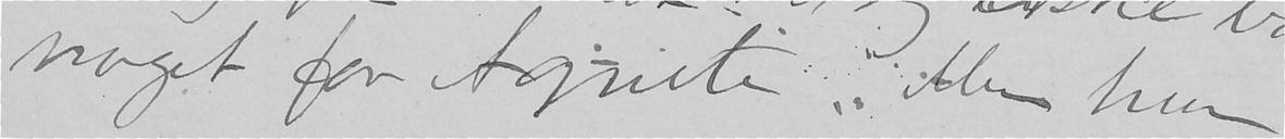noget for Agnete Men hun
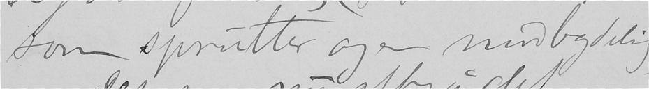som sprutter og er modbydelig)
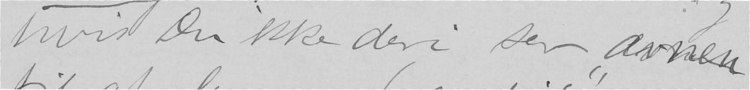hvis Du ikke deri ser "ævnen
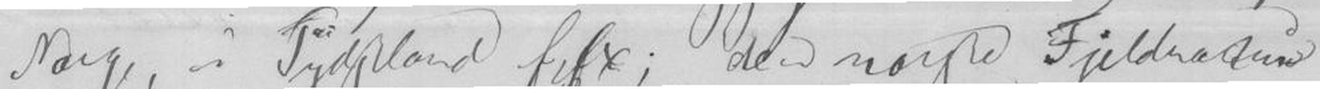Norge, i Tydskland f.Ex; den norske Fjeldnaturen
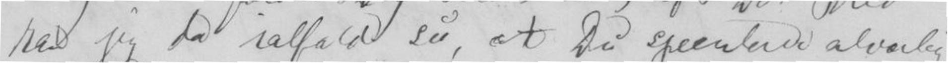kan jeg da ialfald see, at Du speculerer alvorlig
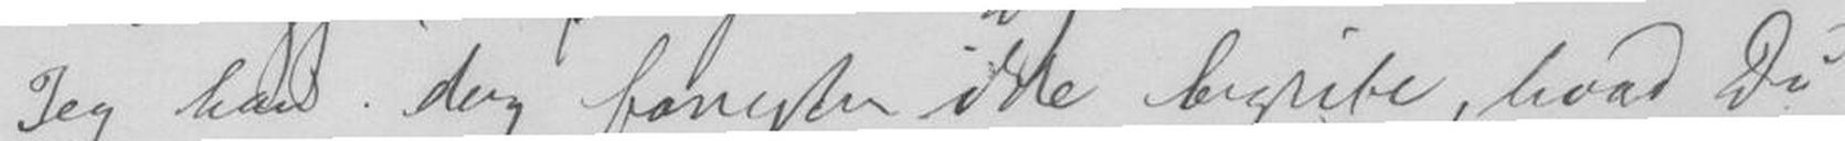Jeg kan deraf forresten ikke begribe, hvad Du
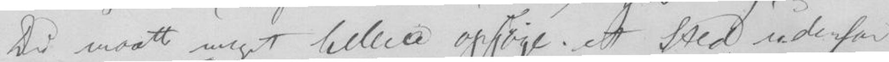Du maatte meget hellere opsøge et Sted udenfor
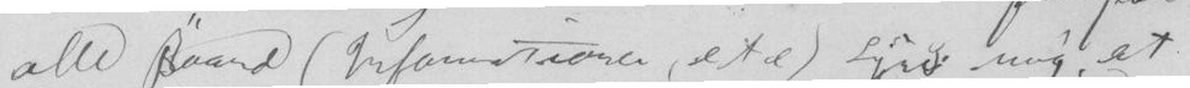alle Baand (Informationer, etc) siger mig, at
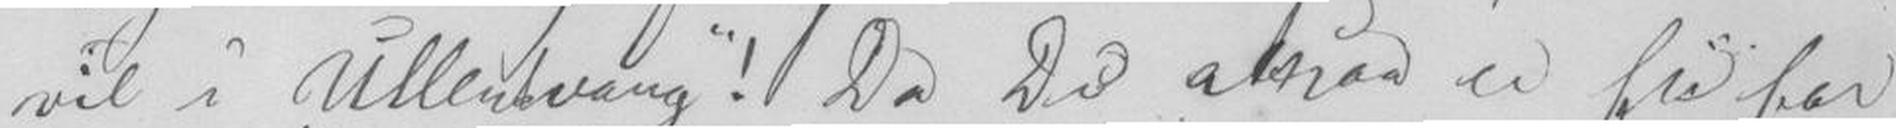vil i Ullensvang! Da Du altsaa er fri for
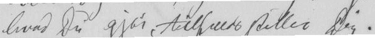hvad Du gjør, tilfredsstiller Dig.
